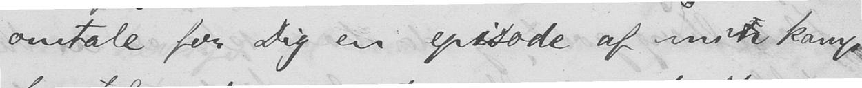omtale for Dig en episode af min kamp
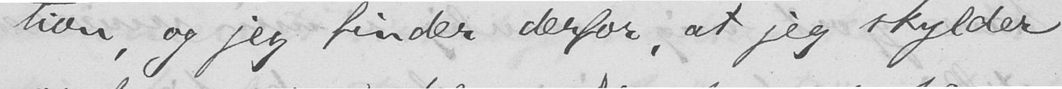tion, og jeg finder derfor, at jeg skylder
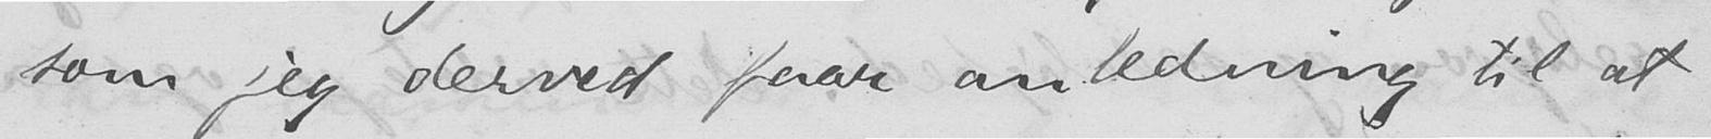som jeg derved faar anledning til at
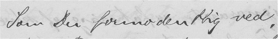Som Du formodentlig ved,
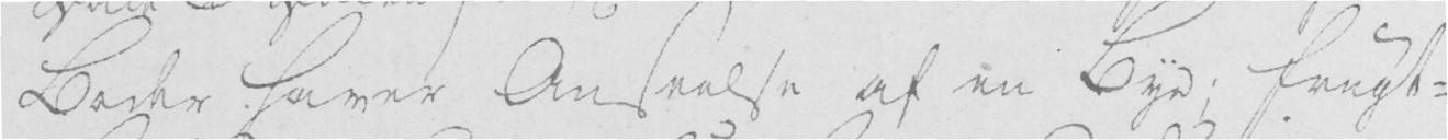Boder haver anseelse af en Bye; frugt-
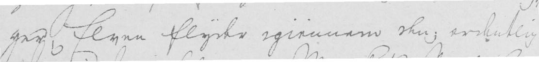ger, Elven flyder igiennem den; ordentlig
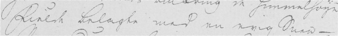Fielde belagte med en evig Snee -
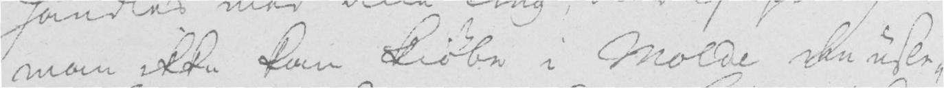man ikke kan kiøbe i Molde den usle-
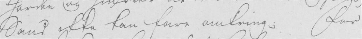Sand ikke kan fare omkring; For
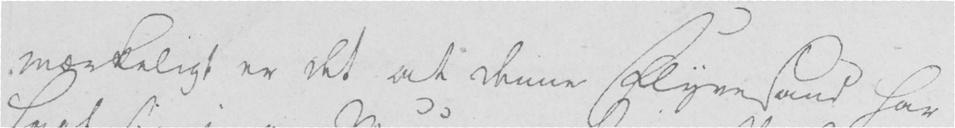mærkeligt er det at denne Flyvesand har
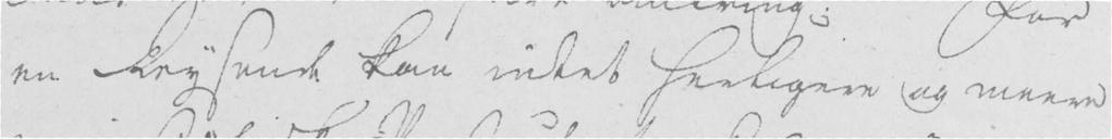en Reysende kan intet herligere og meere
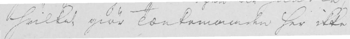hvilket giør Tænkemaaden her ikke
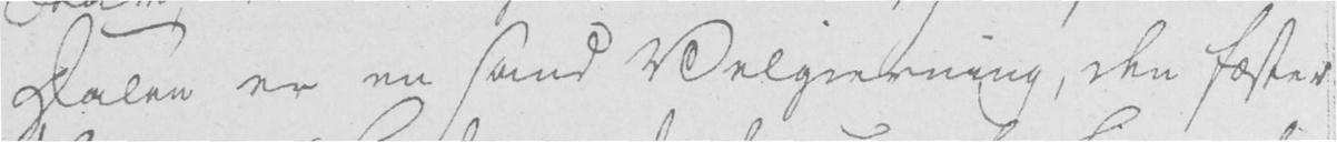Dalen er en sand Velgierning, den fæster
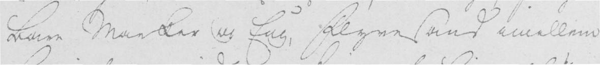bare Marker og Eng, Flyvesand imellem
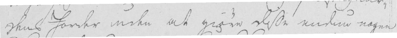dens Jorder uden at giøre disse endnu nogen
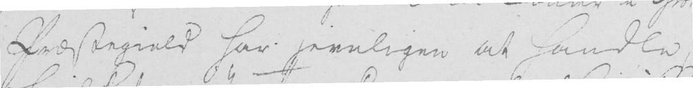Præstegield har jevnligen at handle
